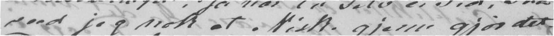veed jeg nok at Niske gjerne gjør det.
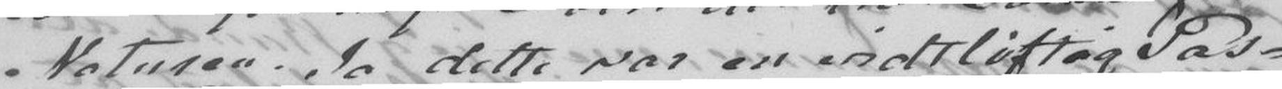Naturen. Ja dette var en vidtløftig Pas-
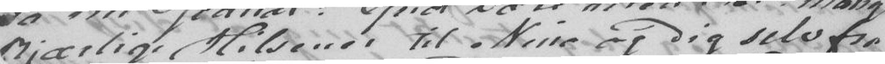kjærlige Hilsener til Nina og Dig selv fra
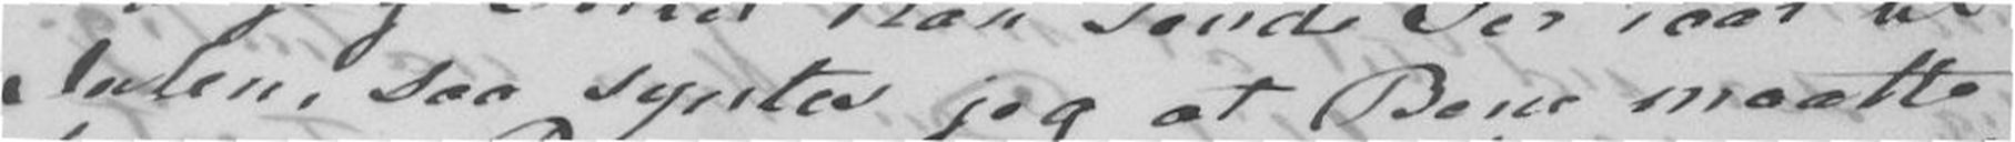Julen, saa syntes jeg at Bene maatte
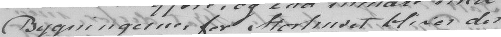Bygningerne, for Storhuset bliver der
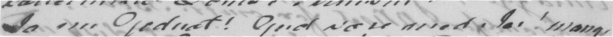Ja nu Godnat! Gud være med Jer! mange
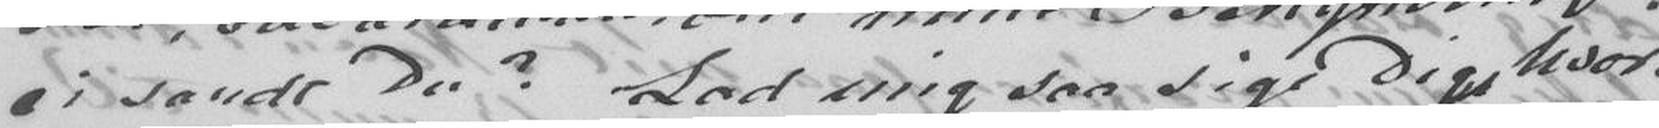ei sandt Du? Lad mig saa sige Dig, hvor-
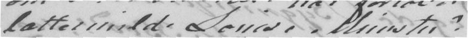lattermilde Louise Münster?
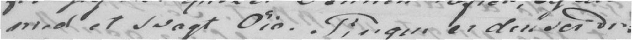med et svagt Øie. Tingen er den ser Du.
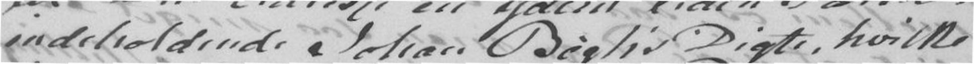indeholdende Johan Bøglis Digte, hvilket
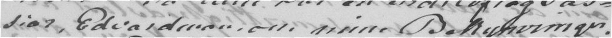siar, Edvardman, om mine Bekymringer
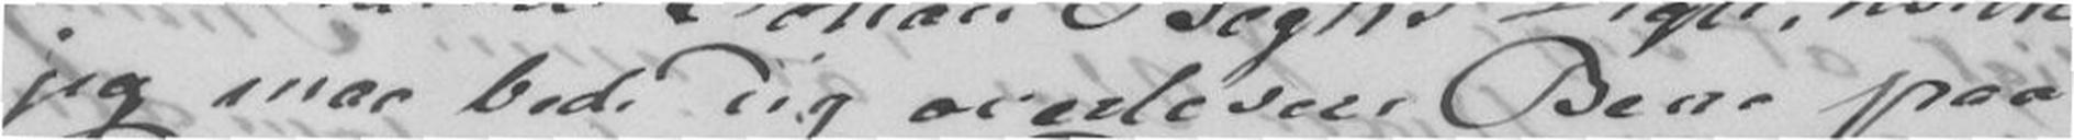jeg maa bede Dig overlevere Bene paa
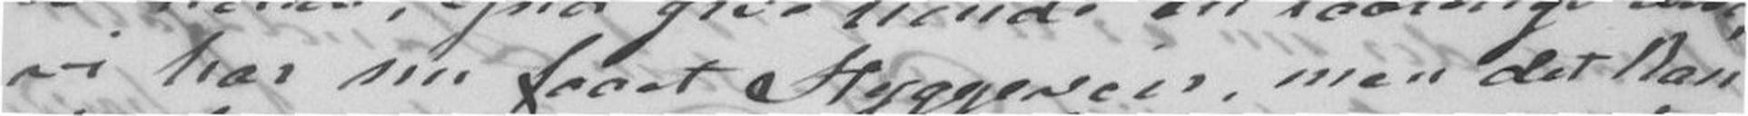vi har nu faaet Styggeveir, men det kan
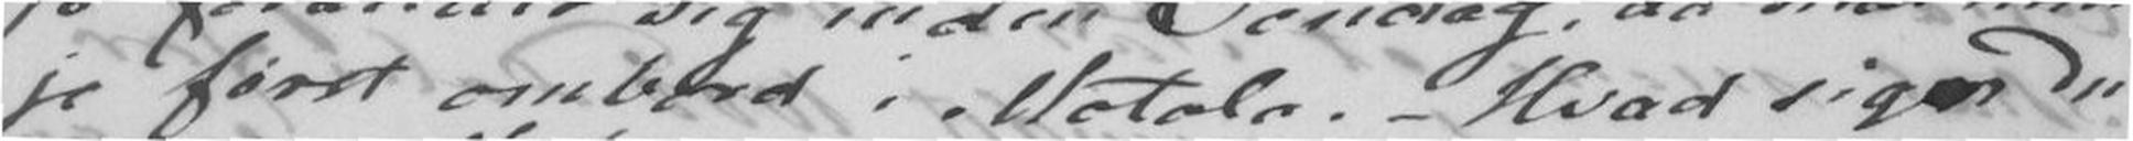jo først ombord i Motala. - Hvad siger Du
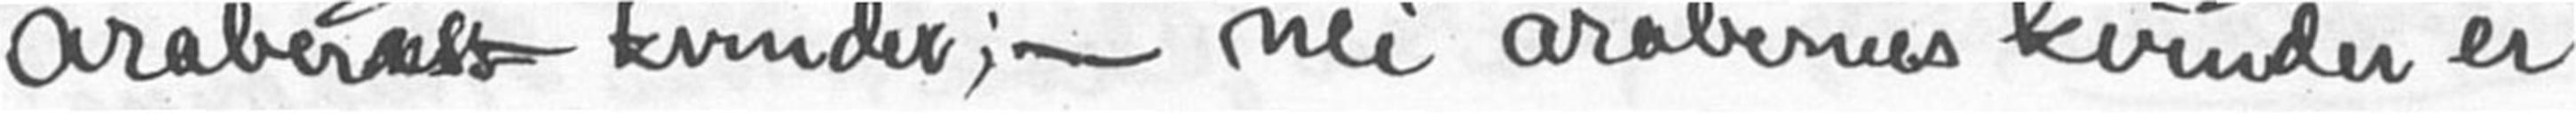arabernes kvinder; - nei arabernes kvinder er
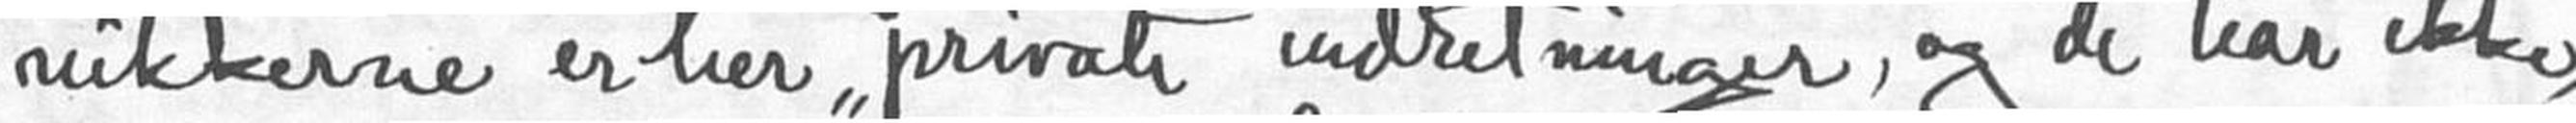nikkerne er her "private indretninger", og de har ikke,
